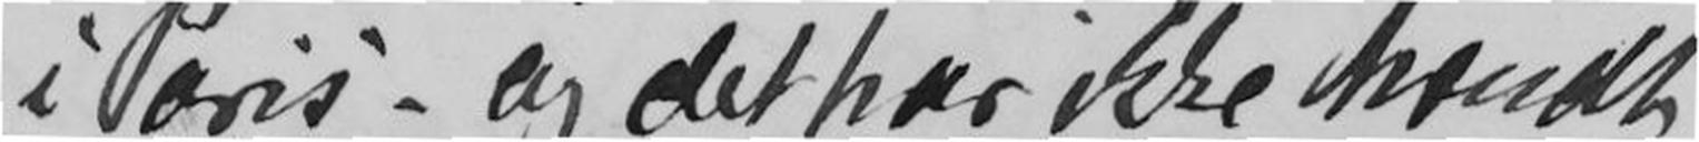i Paris - og det har ikke hændt
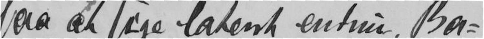saa at sige latent endnu, Ba-
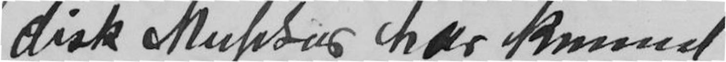disk Musiker har kunnet
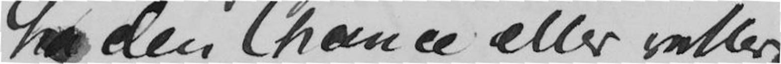ha den Chance eller roller
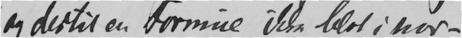og dertil en Formue ikke blot i nor-
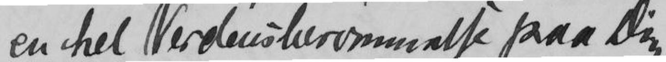en hel Verdensberømmelse paa Dig
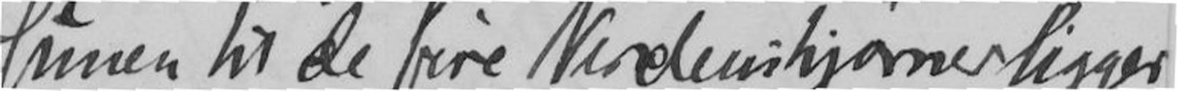sunen til De fire Verdenshjørner ligger
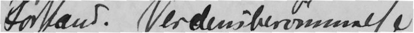Forstand. Verdensberømmelse
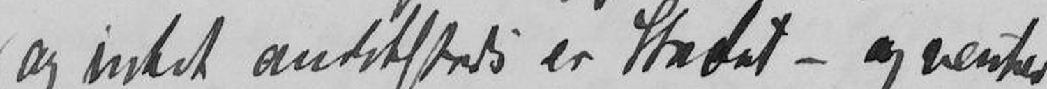og intet andetsteds er Stedet - og venter
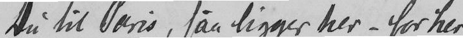Du til Paris, saa ligger her - for her
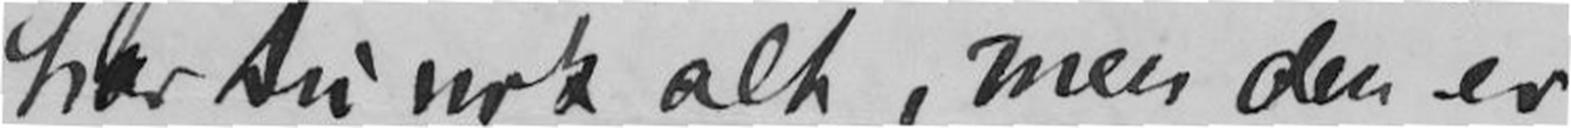har Du nok alt, men den er
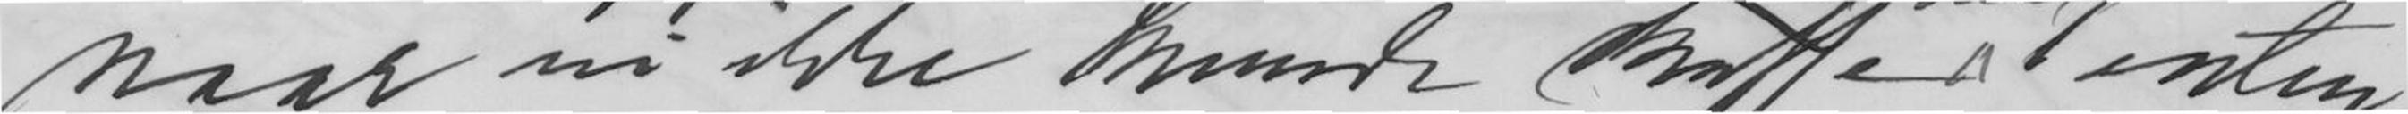naar vi ikke kunde skaffe Dem Texten
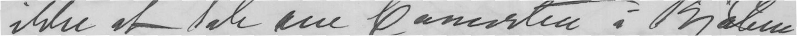ikke at tale om Concerten i Kjøben-
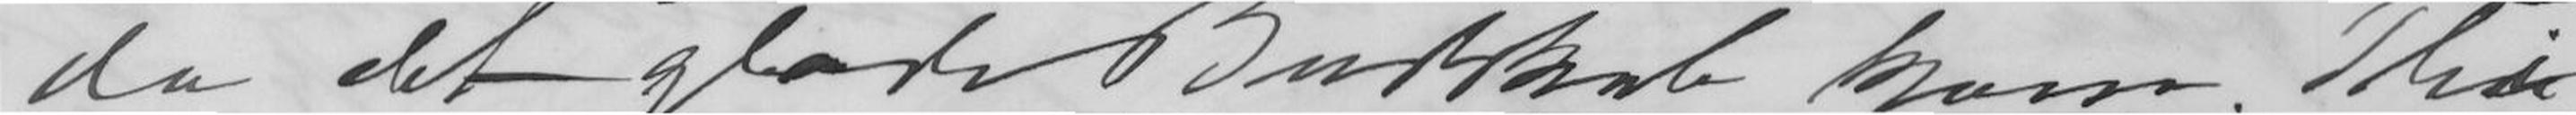da det glade Budskab kom, Thi
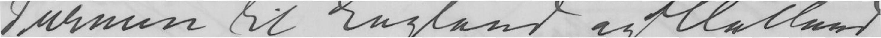Turneen til England og Holland
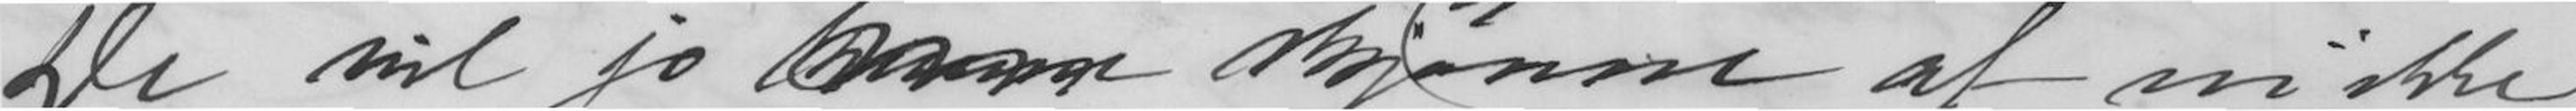De vil jo kun skjønne at vi ikke
